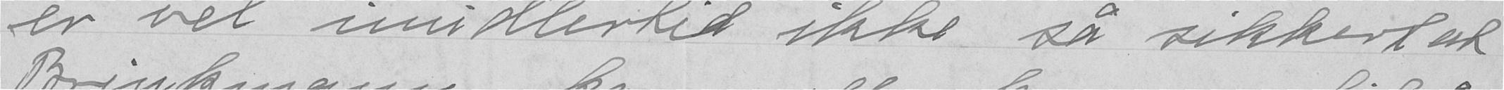er vel imidlertid ikke så sikkert at
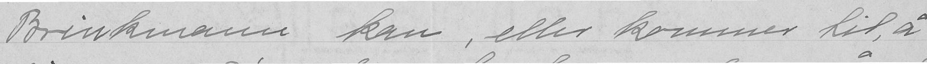Brinkmann kan, eller kommer til, å
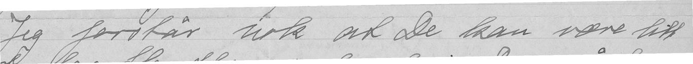Jeg forstår nok at De kan være litt
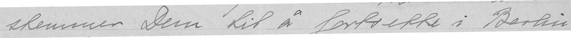stemmer Dem til å fortsette i Berlin
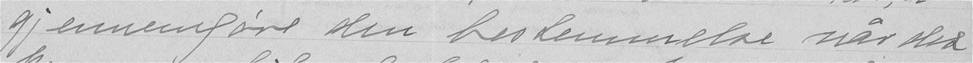gjennemføre den bestemmelse når det
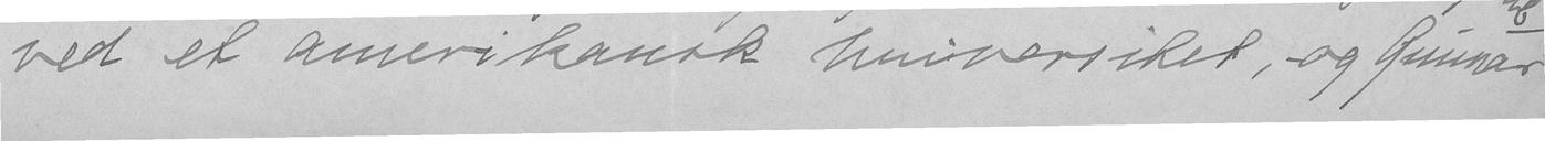ved et amerikansk Universitet, og Gunnar
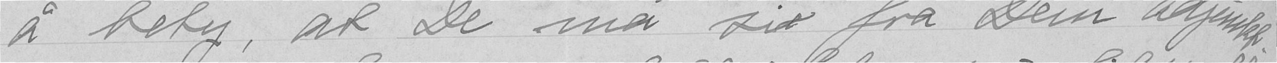å bety, at De må si fra Dem adjunkt
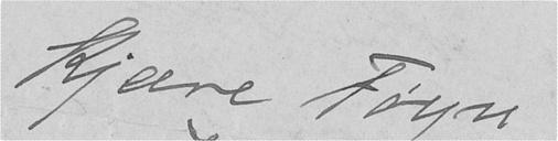Kjære Føyn,
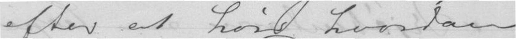efter at høre hvordan
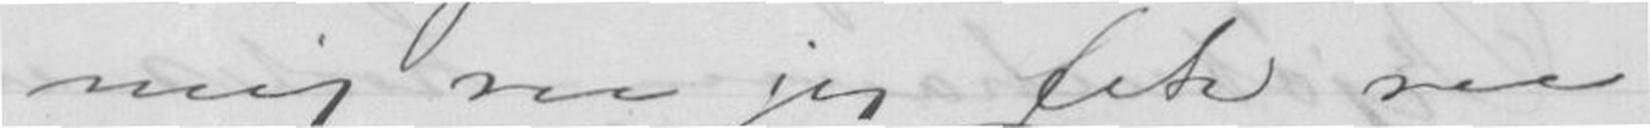mig saa jeg fik see
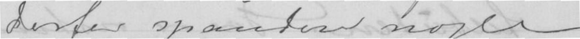derfor spandere nogle
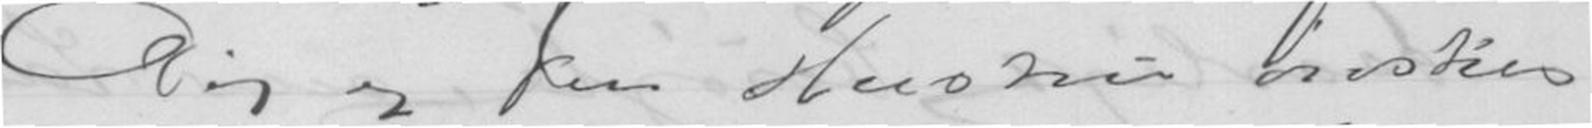Dig og Din Hustru ønskes
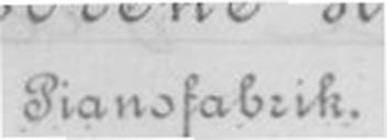Pianofabrik.
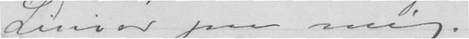Linier paa mig.
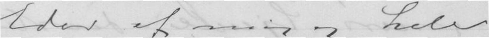Eder af mig og hele
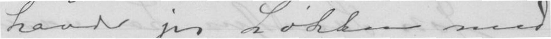havde jeg Løkken med
